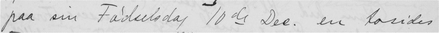paa sin Fødselsdag 10de Dec. en tosides
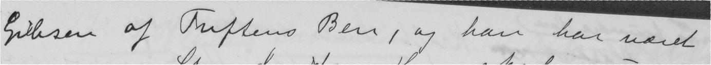Gibsen af Tuftens Ben, og han har været
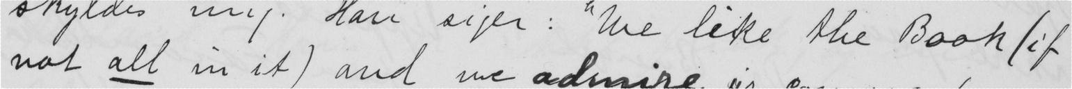skyldes mig. Han siger: "We like the Book (if
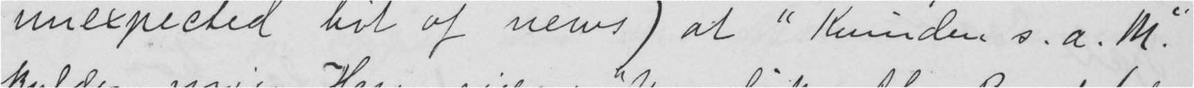unexpected bit of news) at "Kvinden s. a. M".
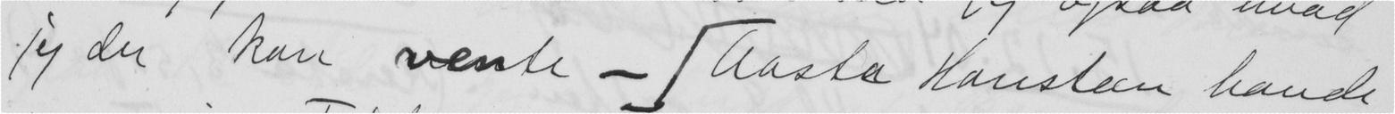jeg der kan vente - Aasta Hansteen havde
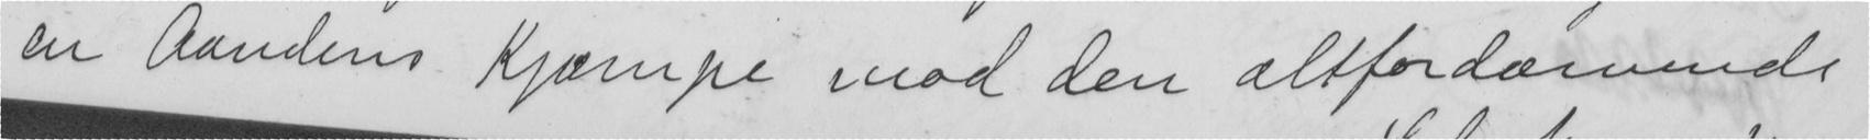en Aandens Kjæmpe mod den altfordærvende
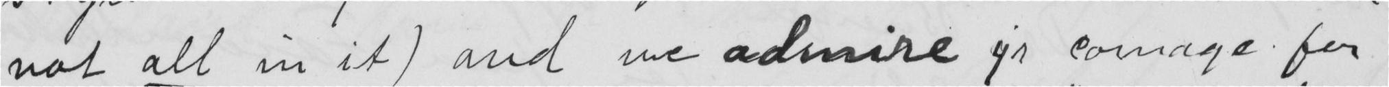not all in it) and we admire y'r courage for
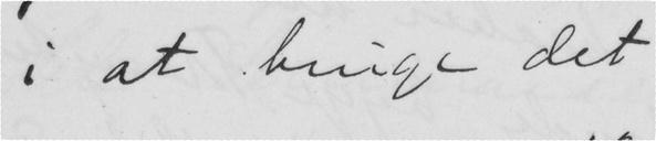i at bruge det
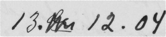13. 12.04
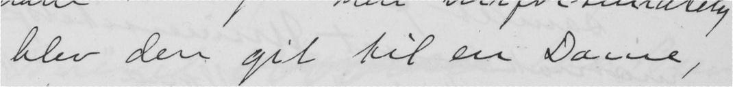blev den git til en Dame,
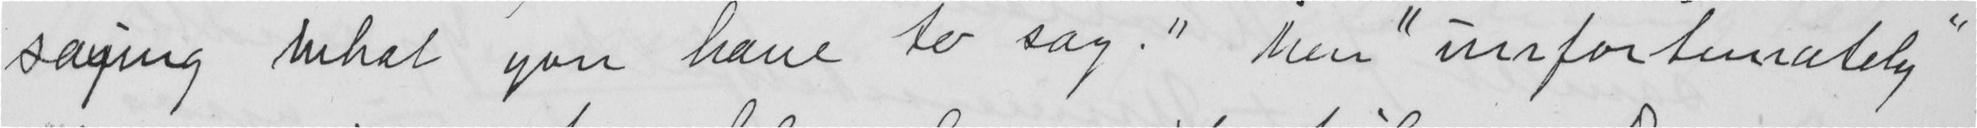saying what you have to say." Men "unfortunately"
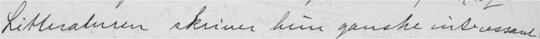Litteraturen skriver hun ganske interessant
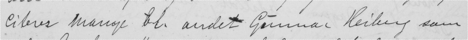citeres Mange bl. andet Gunnar Heiberg som
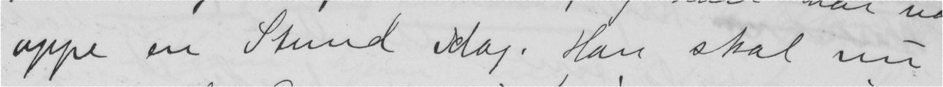oppe en Stund idag. Han skal nu
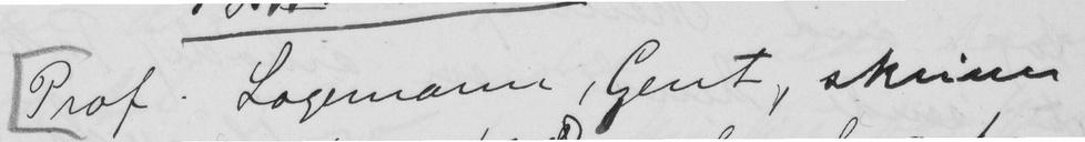Prof. Logemann, Gent, skriver
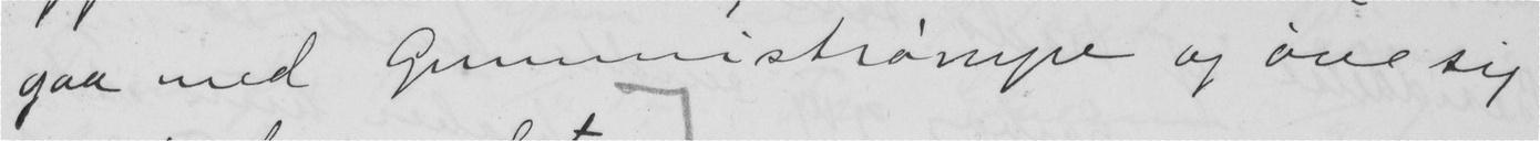gaa med Gummistrømpe og øve sig
